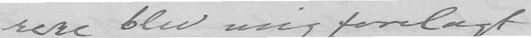rene blev mig forelagt
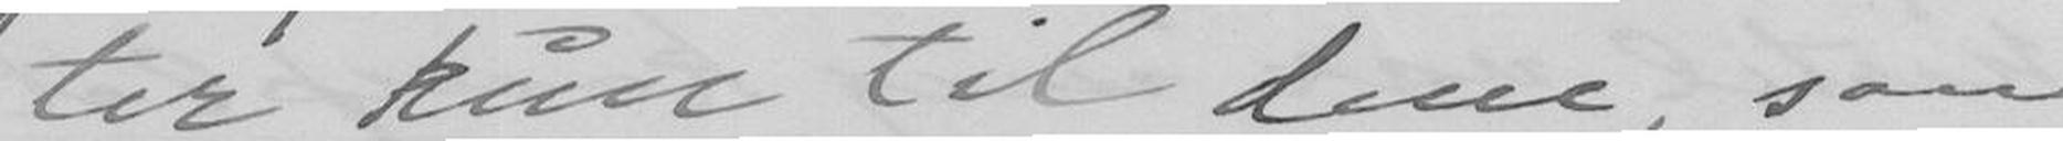ter kun til dem, som
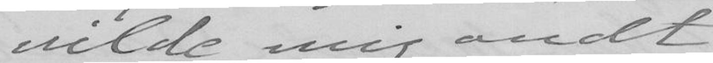vilde mig andet.
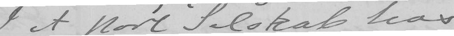I et stort Selskab hos
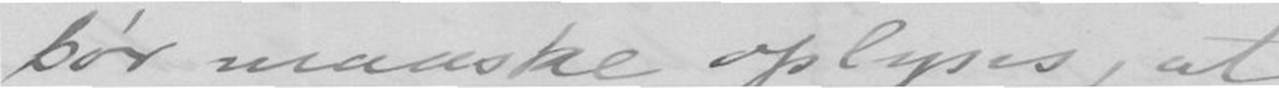bør maaske oplyses, at
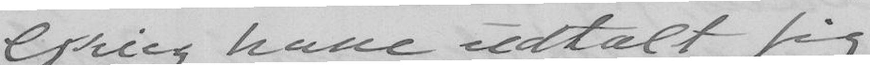Grieg have Udtalt sig
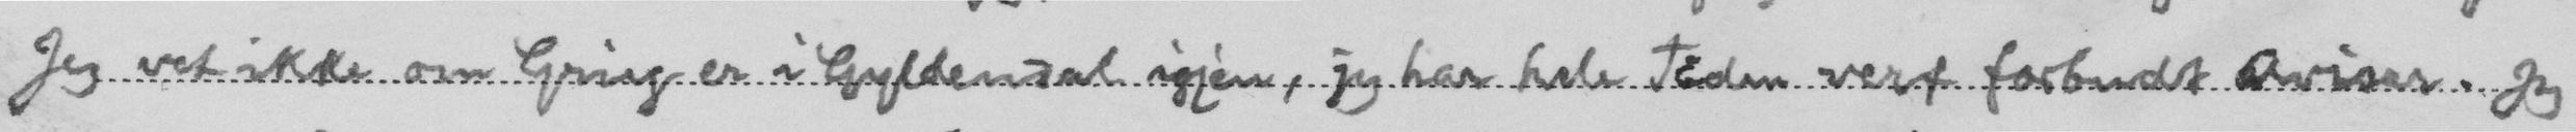Jeg vet ikke om Grieg er i Gyldendal igjen, jeg har hele Tiden vert forbudt Aviser. Jeg
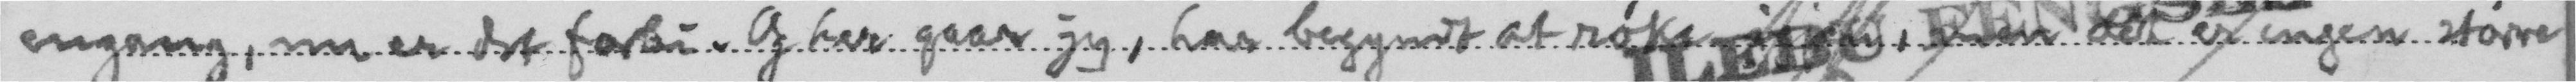engang, nu er det forbi. Og her gaar jeg, har begyndt at røke igjen, men det er ingen større
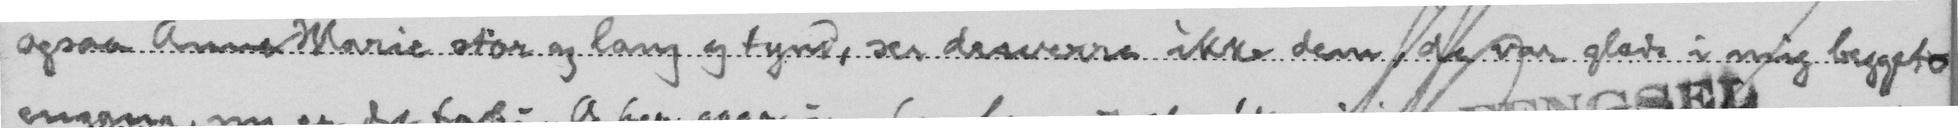ogsaa Anne Marie stor og lang og tynd, ser desverre ikke dem, de var glade i mig beggeto
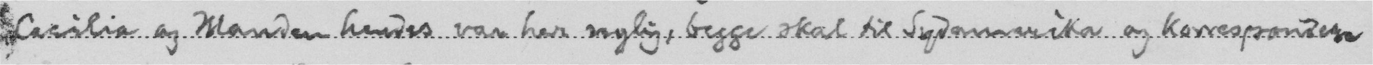Cecilia og Manden hendes var her nylig, begge skal til Sydamerika og korrespondere
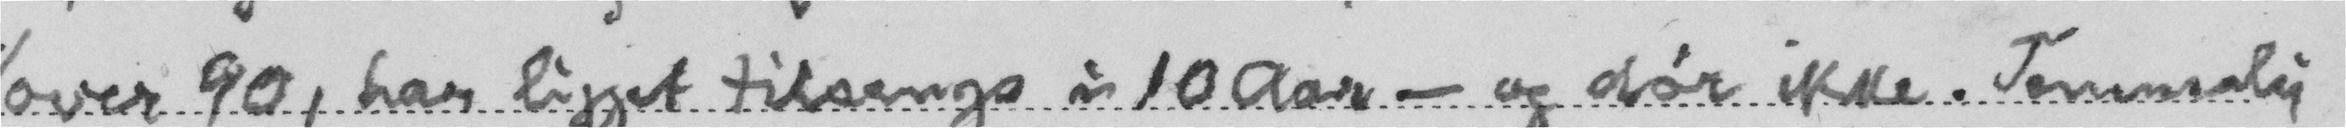over 90, har ligget tilsengs i 10 Aar - og dør ikke. Temmelig
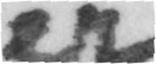er
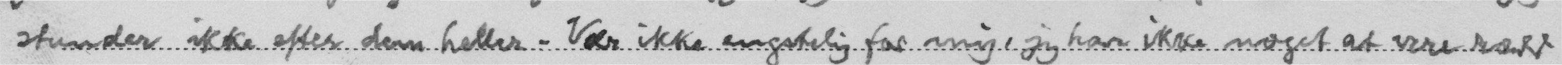stunder ikke efter dem heller. Vær ikke engstelig for mig, jeg har ikke noget at vere rædd
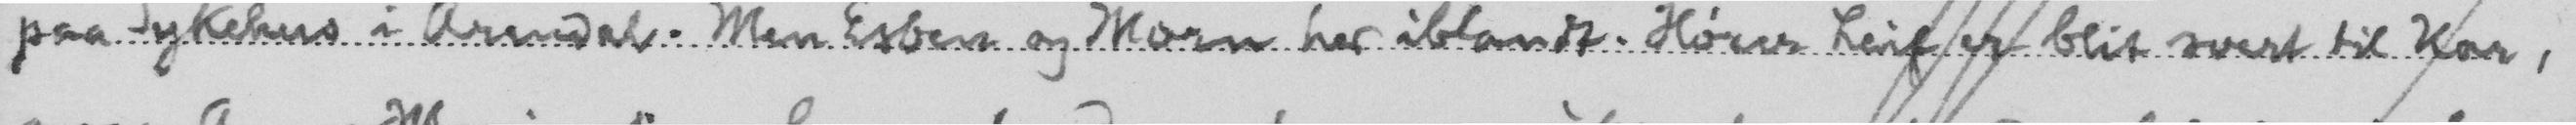paa Sykehus i Arendal. Men Esben og Morn her iblandt. Hører Leif er blit svert til Kar,
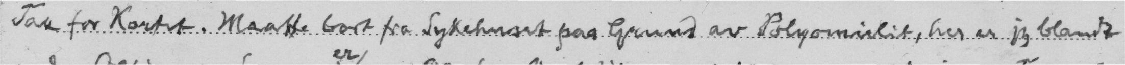Tak for Kortet. Maatte bort fra Sykehuset paa Grund av Polyomielit, her er jeg blandt
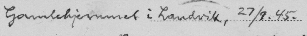Gamlehjemmet i Landvik, 27/9. 45.
